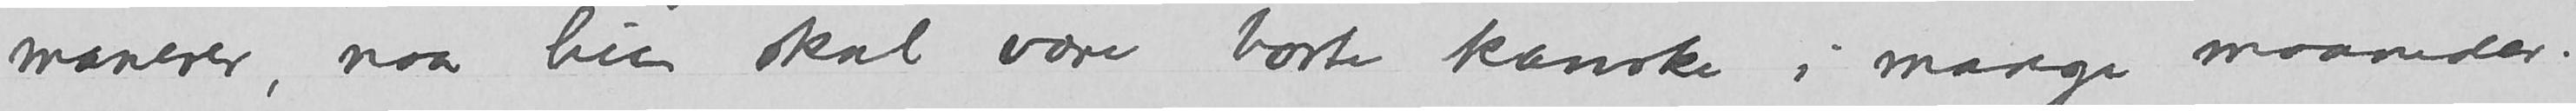manerer, naar hun skal være borte kanske i mange maaneder.
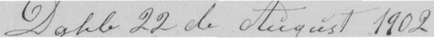Dahle 22de August 1902
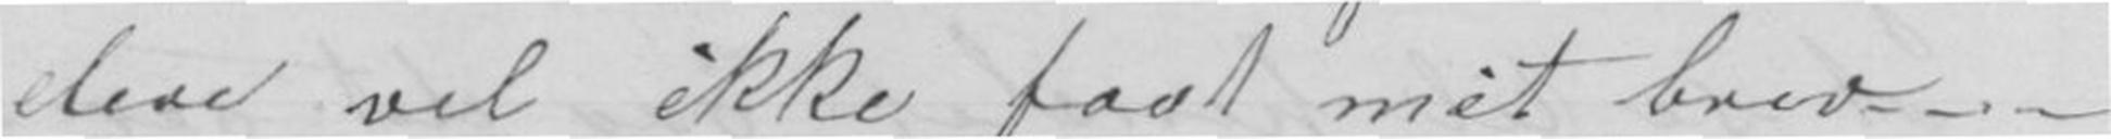dere vel ikke faat mit brev - - -
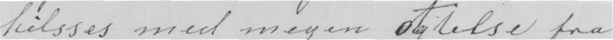hilsses med megen agtelse fra
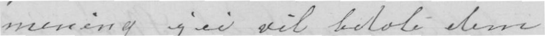mening jei vil betole dem
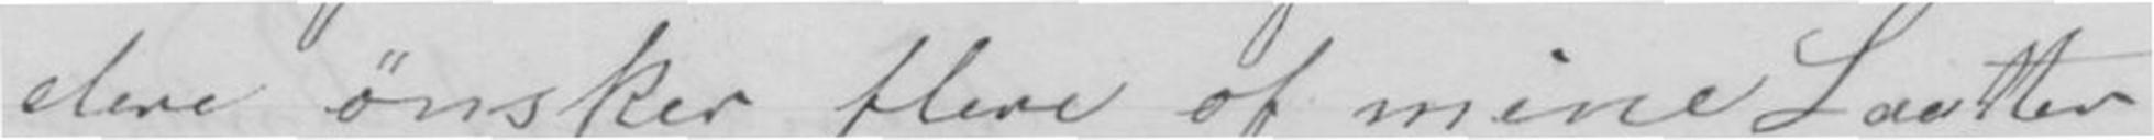dere ønsker flere of mine Laatter
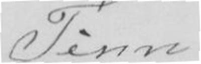Tinn
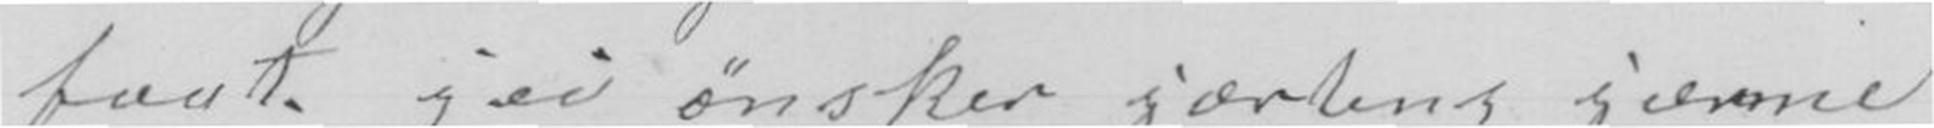faat. jei ønsker jærtens jærne
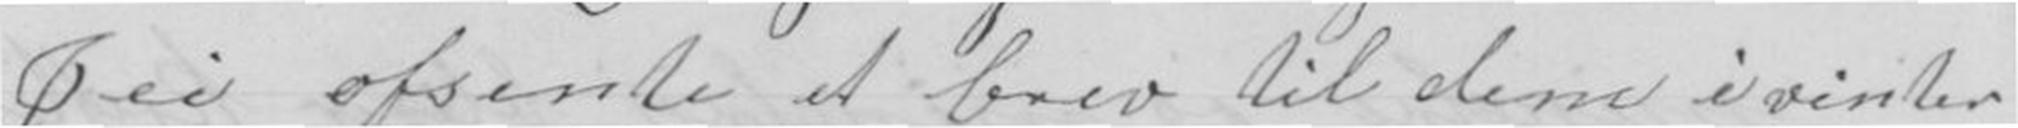Jei ofsente et brev til dem i vinter
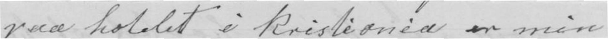paa hotelet i kristiania er min
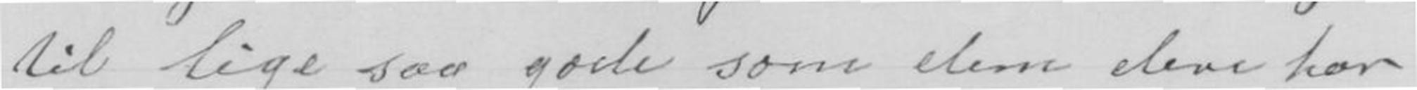til lige saa gode som dem dere har
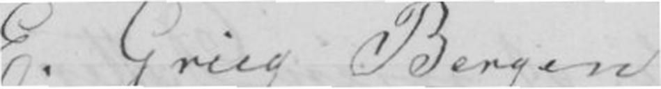E. Grieg Bergen
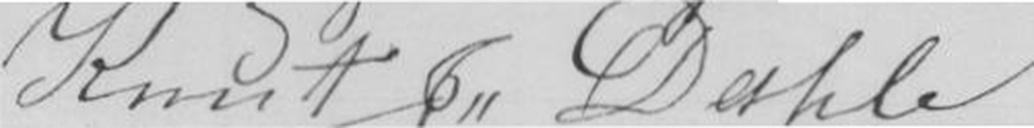Knut J. Dahle
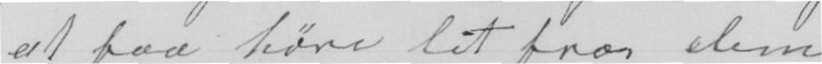at faa høre lit fro dem
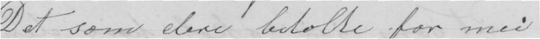Det som dere betolte for mei
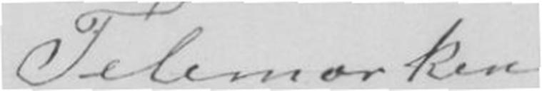Telemarken
